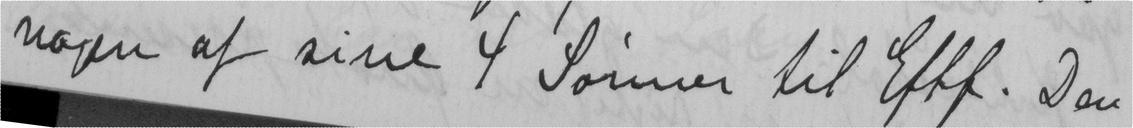nogen af sine 4 Sønner til Eftf. Den
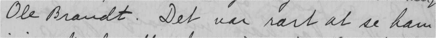Ole Brandt. Det var rart at se ham
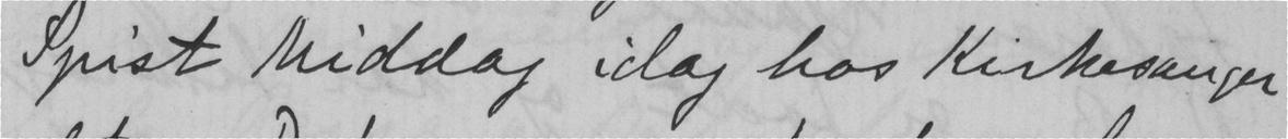Spist Middag idag hos Kirkesanger
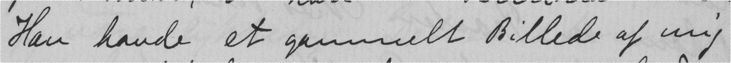Han havde et gammelt Billede af mig
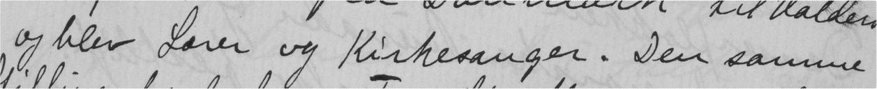og blev Lærer og Kirkesanger. Den samme
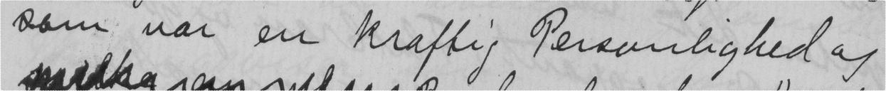som var en kraftig Personlighed og
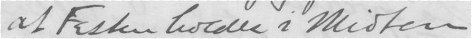at Festen holdes i Midten
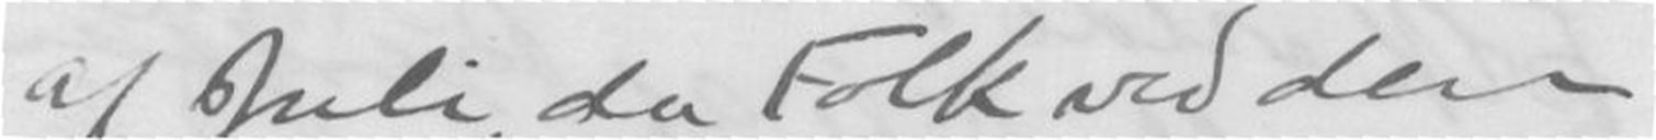af Juli, da Folk ved den
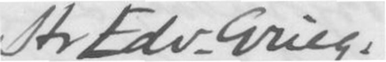Hr Edv. Grieg.
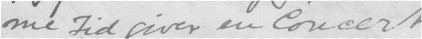me Tid giver en Concert
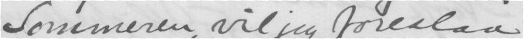Sommeren, vil jeg foreslaa
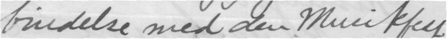bindelse med den Musikfest
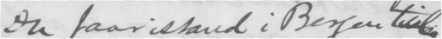Du faar istand i Bergen til
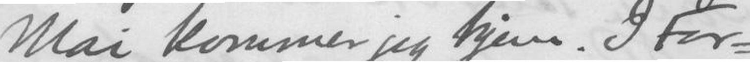Mai kommer jeg hjem. I For-
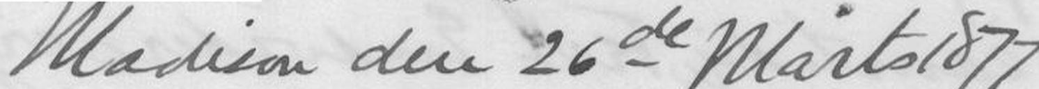Madison den 26de Marts 1877
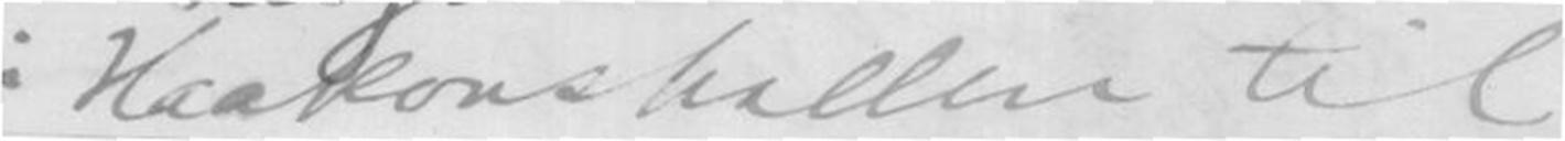i Haakonshallen til
